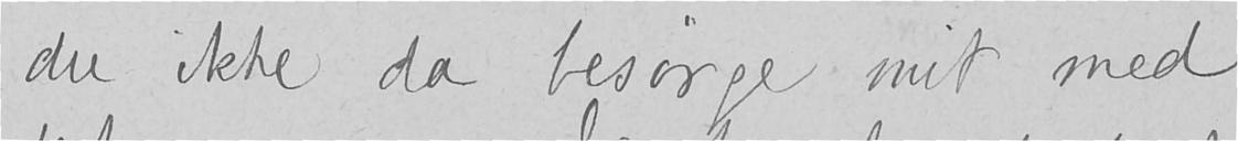du ikke da besørge mit med
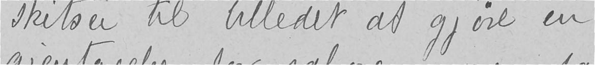skitser til billedet at gjøre en
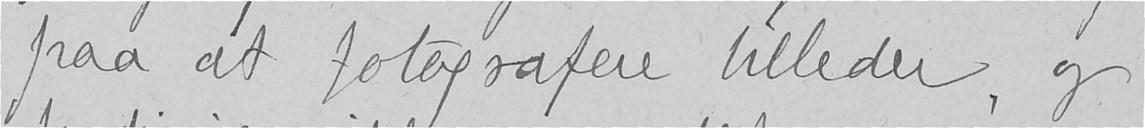paa at fotografere billeder, og
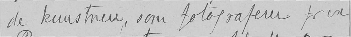de kunstnere, som fotograferer frex.
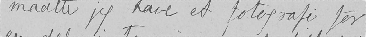maatte jeg have et fotografi for
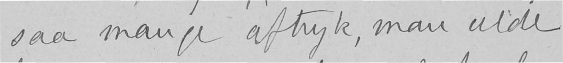saa mange aftryk, man vilde
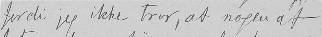fordi jeg ikke tror, at nogen af
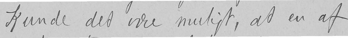Kunde det være muligt, at en af
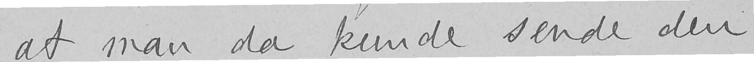at man da kunde sende den
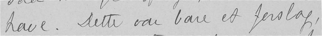have. Dette var bare et forslag,
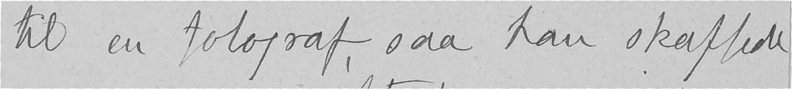til en fotograf, saa han skaffede
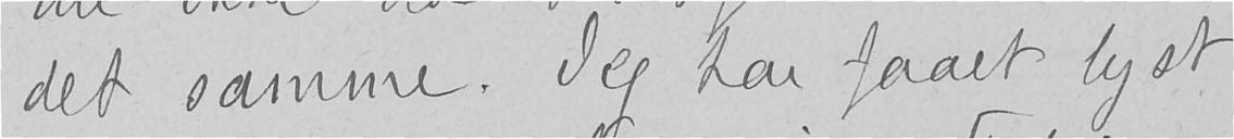det samme. Jeg har faaet lyst
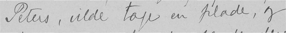Peters, vilde tage en plade, og
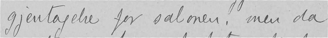gjentagelse for salonen, men da
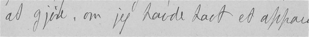at gjøre, om jeg havde havt et appar
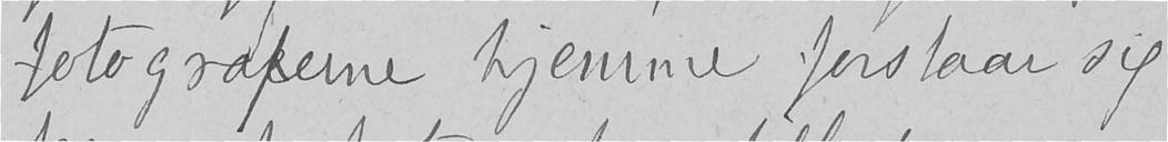fotograferne hjemme forstaar sig
